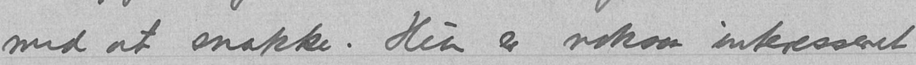med at snakke. Hun er noksaa interesseret
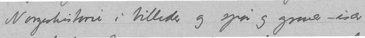Norgeshistorie i billeder og spør og graver – især
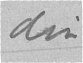din
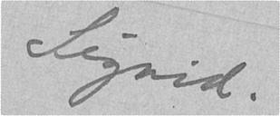Sigrid.
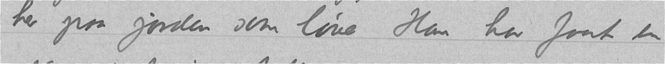her paa jorden som løve.  Han har faat en
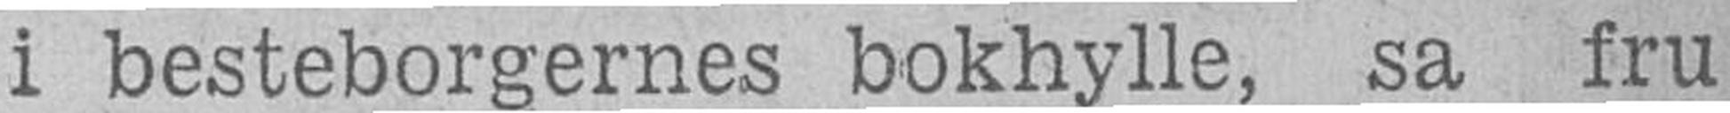i besteborgernes bokhylle, sa fru
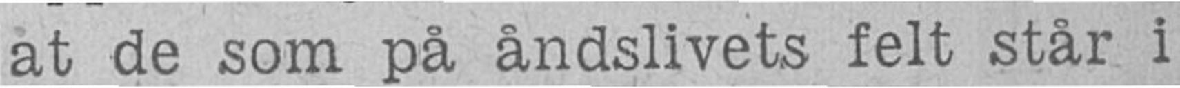at de som på åndslivets felt står i
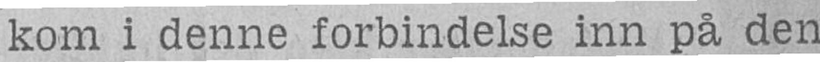kom i denne forbindelse inn pâ den
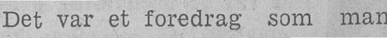Det var et foredrag som man
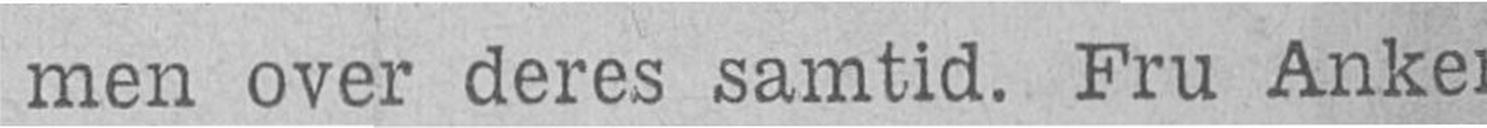men over deres samtid. Fru Anker
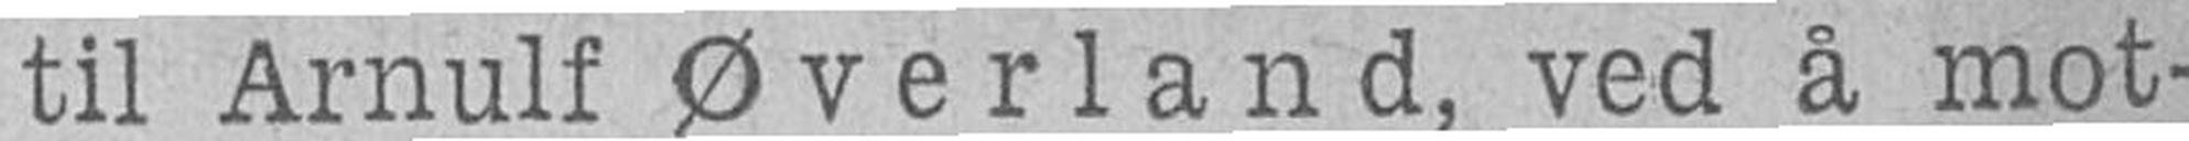til Arnulf Øverland, ved å mot-
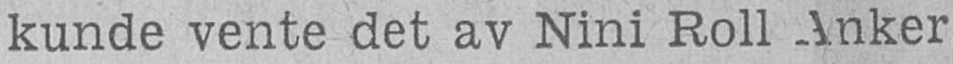kunde vente det av Nini Roll Anker,
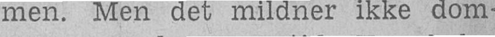men. Men det mildner ikke dom-
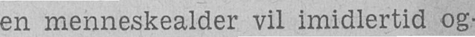en menneskealder vil imidlertid og-
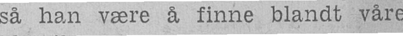så han være å finne blandt våre
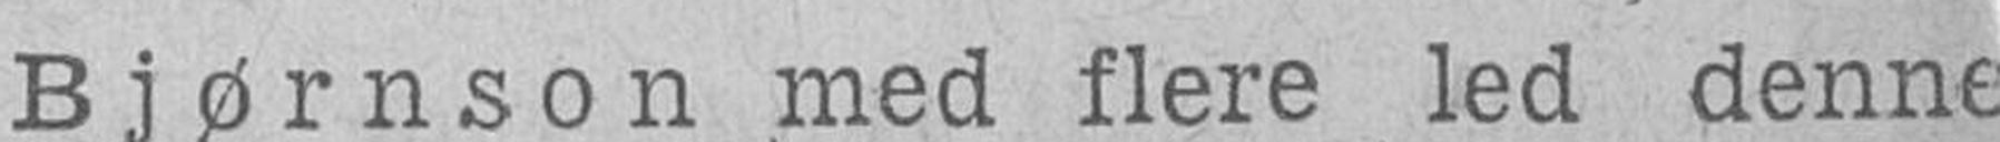Bjørnson med flere led denne
